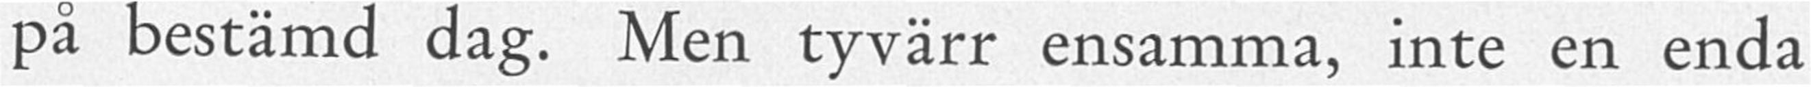på bestämd dag. Men tyvärr ensamma, inte en enda
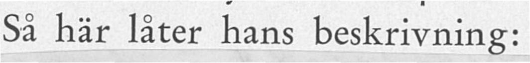Sa här låter hans beskrivning:
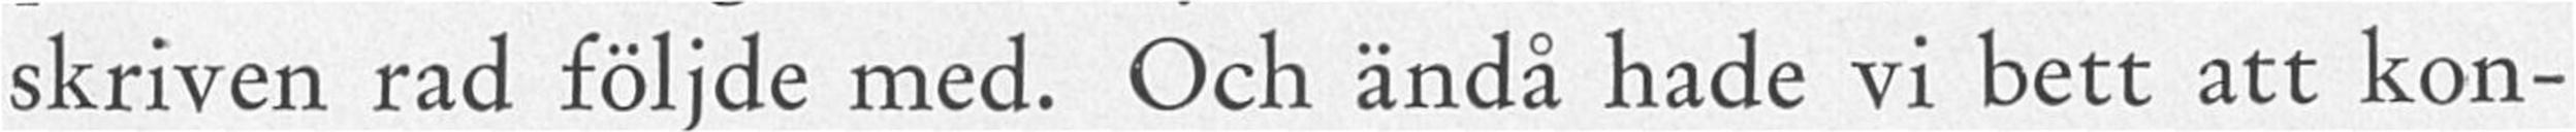skriven rad följde med. Och ändå hade vi bett att kon-
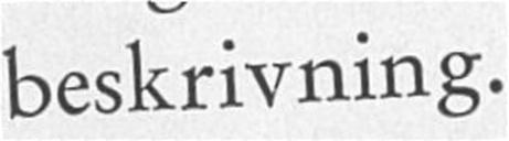beskrivning.
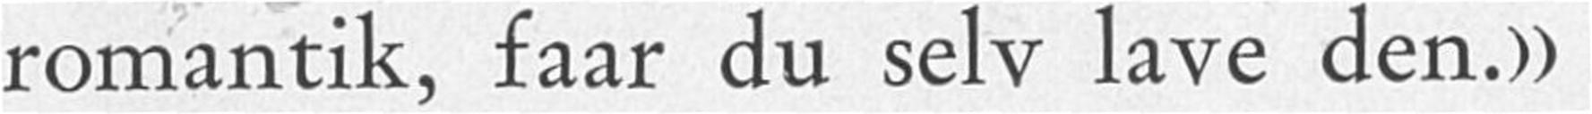romantik, faar du selv lave den."
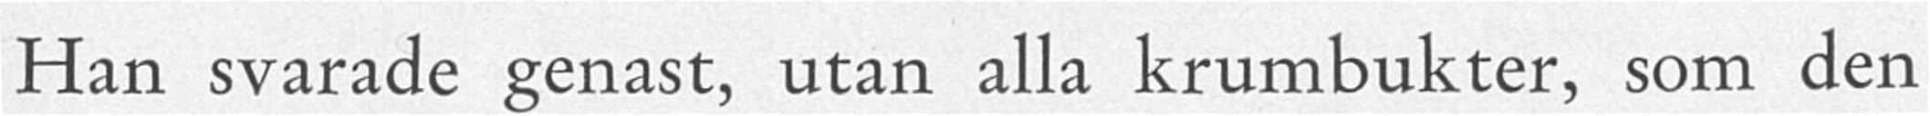Han svarade genast, utan alla krumbukter, som den
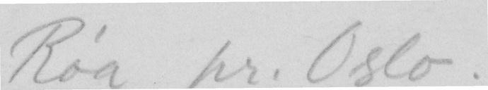Røa pr. Oslo.
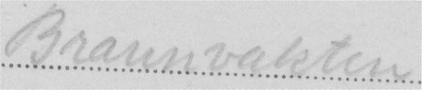Brannvakten
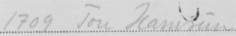1709 Tore Hamsun
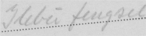Ilebu fengsel
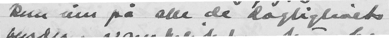kom hun inn på alle de dagliglivets
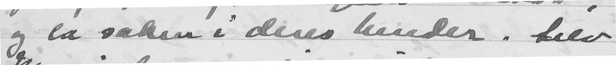og la saken i deres hender. Selv
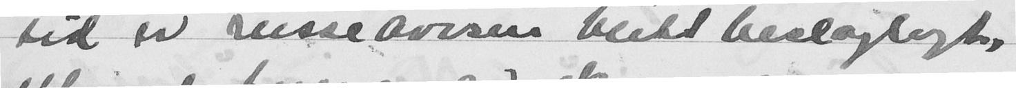tid er russeavisen blitt beslaglagt,
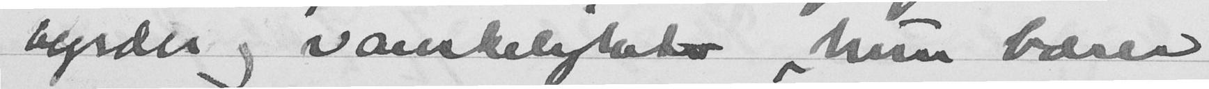byrder og vanskeligheter, hun bærer
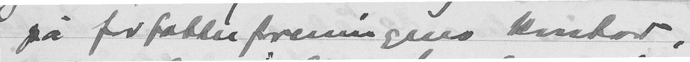på forfatterforeningens kontor,
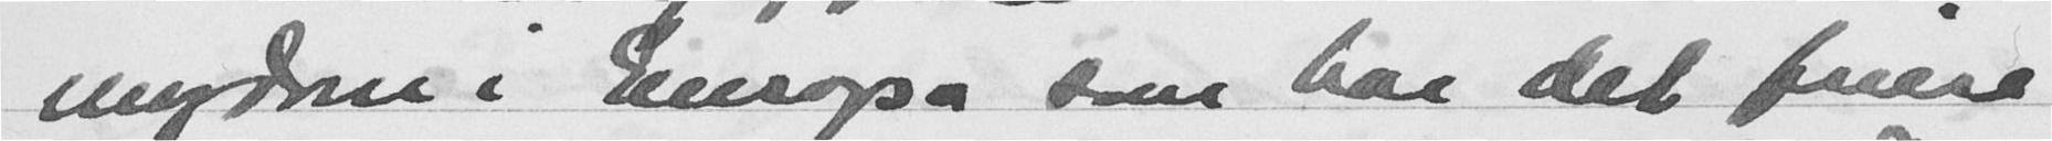ungdom i Europa, som har det friere
